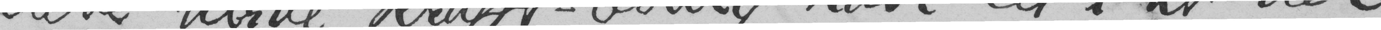dette turde Krafft-Ebing have ret i, at der
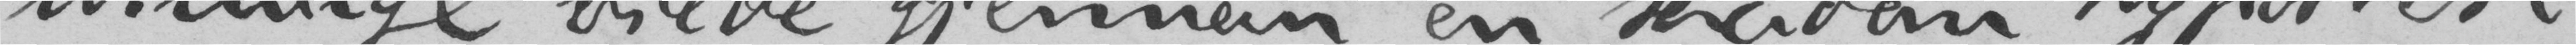urninge vilde gjennem en saadan hypothese
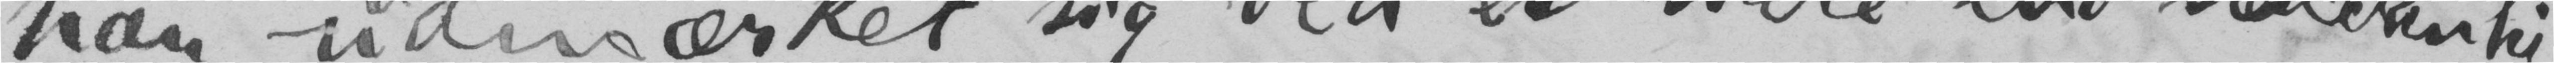har udmærket sig ved et mere en sædvanlig
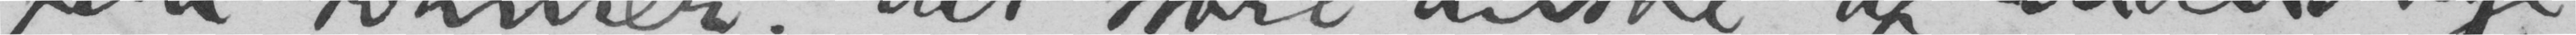faa sønner; det store antal af mandlige
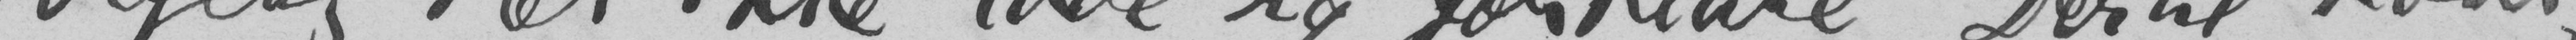følgelig slet ikke lade sig forklare. Dertil kom-
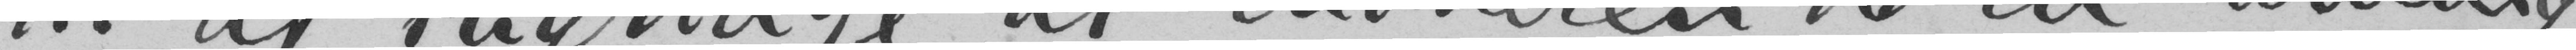til at iagttage, at moderen til en urning
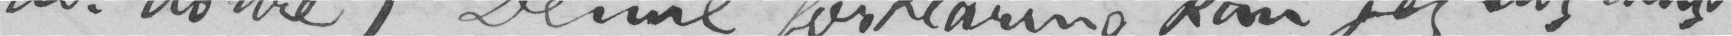do. døttre). Denne forklaring kan jeg dog langt-
